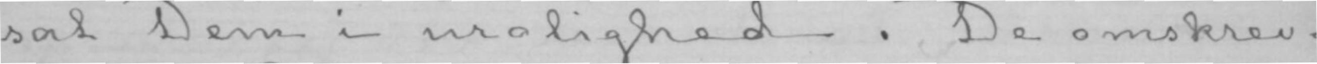sat Dem i urolighed. De omskrev-
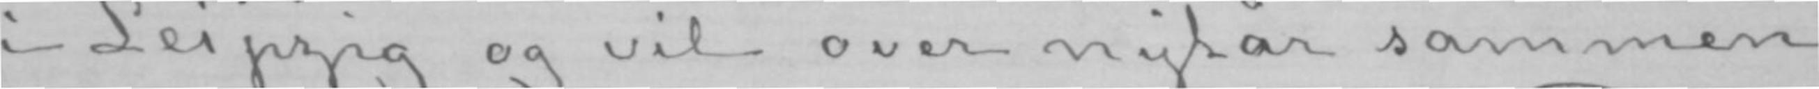i Leipzig og vil over nytår sammen
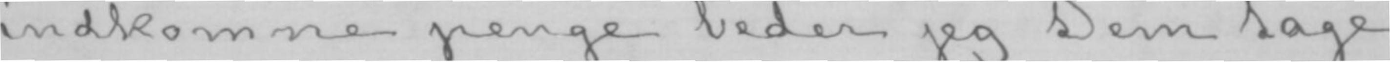indkomne penge beder jeg Dem tage
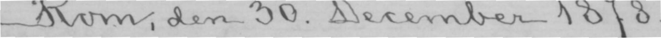Rom, den 30. December 1878.
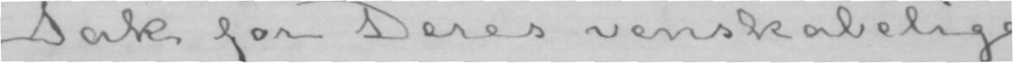Tak for Deres venskabelige
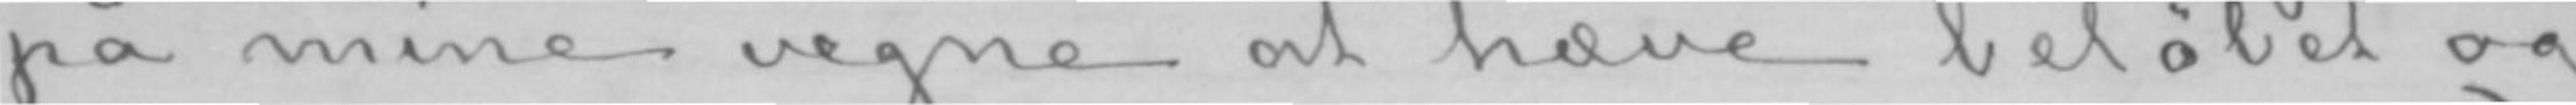på mine vegne at hæve beløbet og
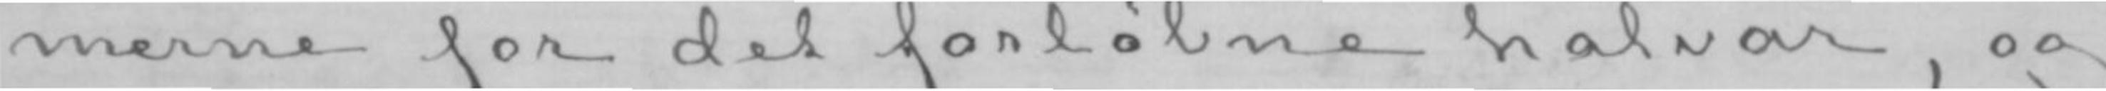merne for det forløbne halvår, og
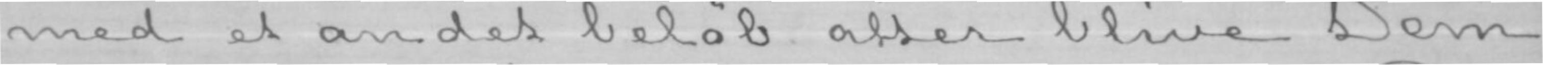med et andet beløb atter blive Dem
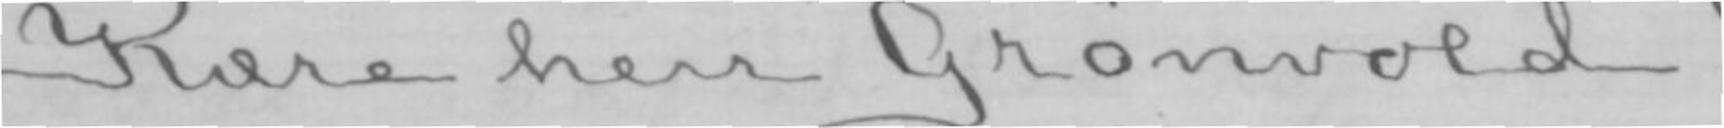Kære herr Grønvold!
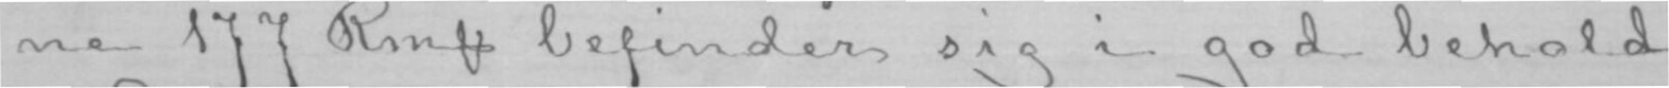ne 177 RmՖ befinder sig i god behold
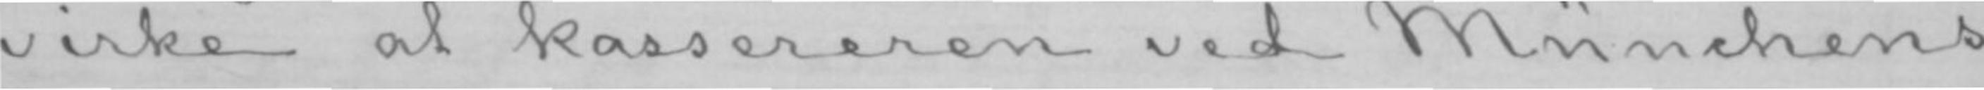virke at kassereren ved Münchens
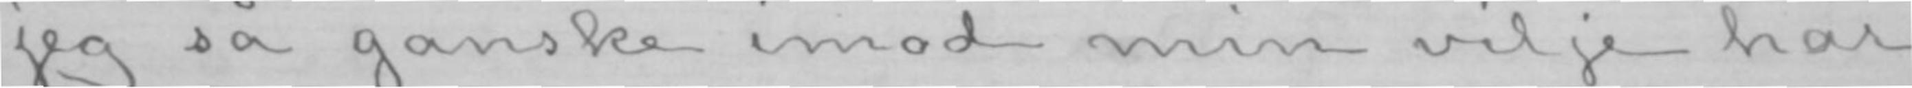jeg så ganske imod min vilje har
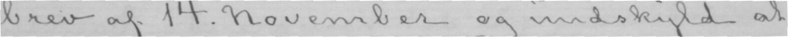brev af 14. November og undskyld at
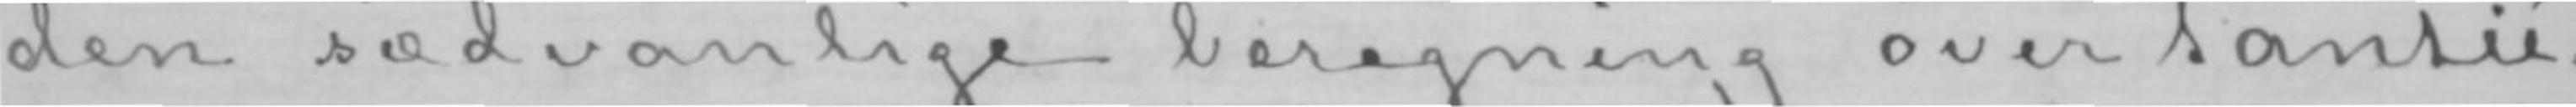den sædvanlige beregning over tantié-
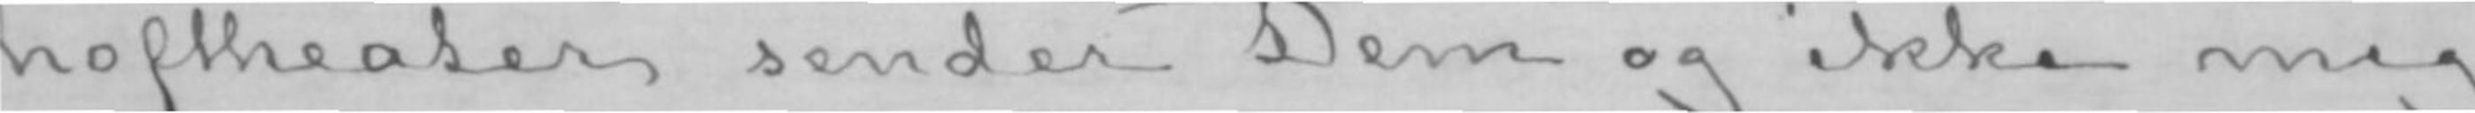hoftheater sender Dem og ikke mig
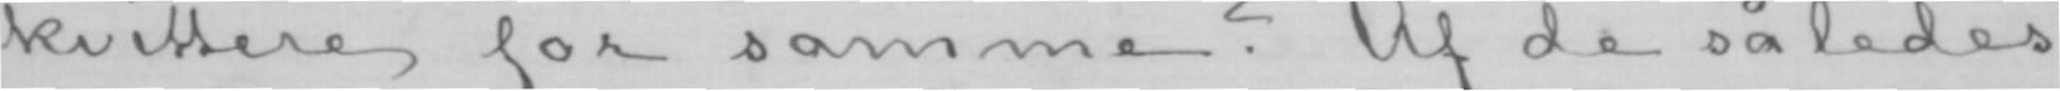kvittere for samme? Af de således
